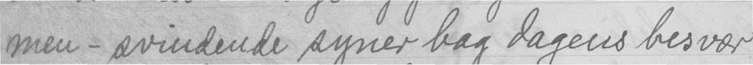men - svindende syner bag dagens besvær
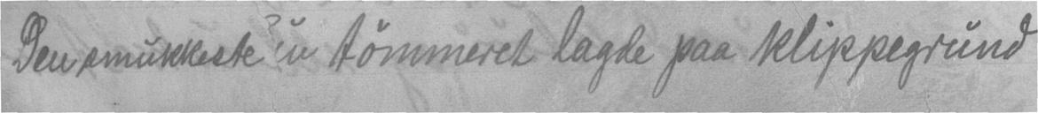Den smukkeste u  tømmeret lagde paa klippegrund
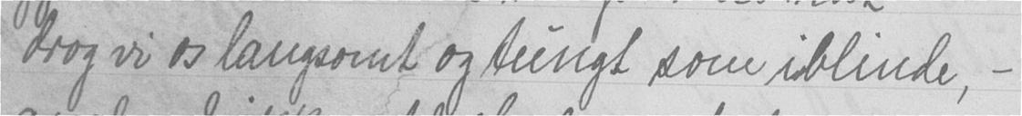drog vi os langsomt og tungt som iblinde, -
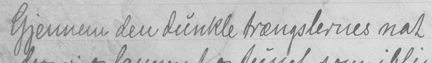Gjennem den dunkle trængslernes nat
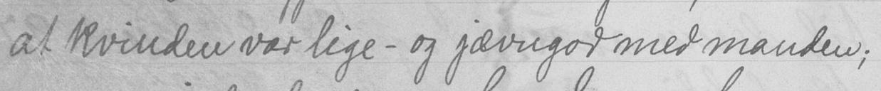at kvinden var lige - og jævngod med manden;
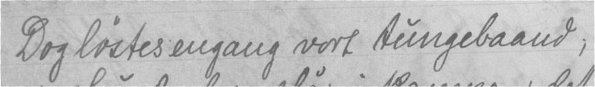Dog løstes engang vort tungebaand;
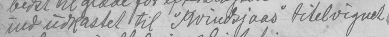ind udkastet til "Kvindsjaas" tilelvignet.
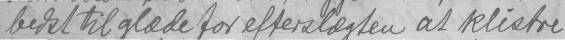bedst til glæde for efterslægten at klistre
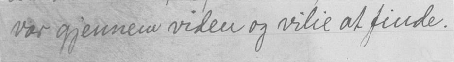var gjennem viden og vilie at finde.
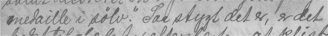medaille i sølv." Saa stygt det er, er det
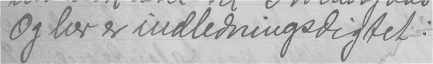Og her er indledningsdigtet:
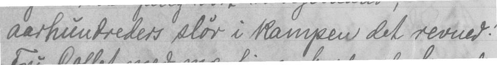aarhundreders slør i kampen det revned!
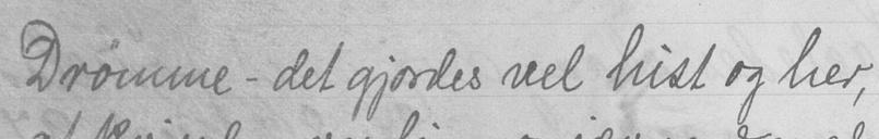Drømme - det gjordes vel hist og her,
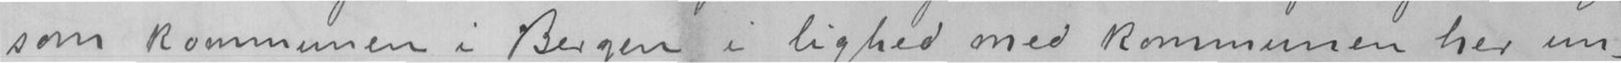som kommunen i Bergen i lighed med kommunen her un-
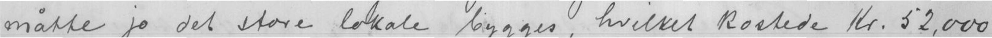måtte jo det store lokale bygges, hvilket Kostede Kr. 52.000
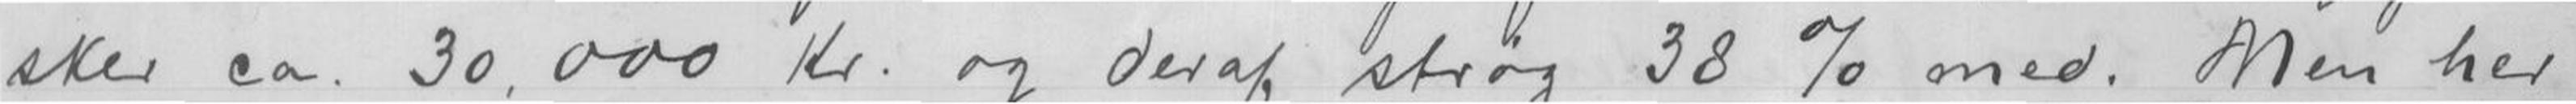sker ca. 30.000 Kr. og deraf strøg 38 % med. Med her
